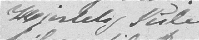Hjertelig Jule
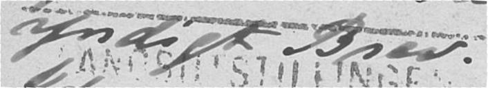yndigt Brev.
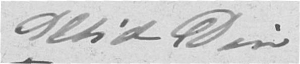Altid Din
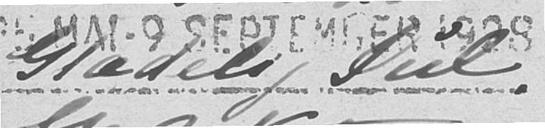Glædelig Jul!
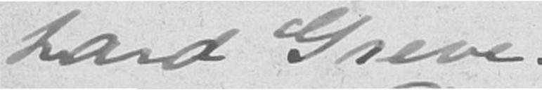hard Greve.
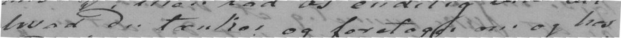hvad Du tænker og foretager om og hos
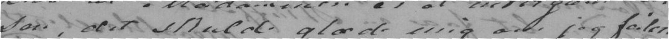sen; det skulde glæde mig om jeg feiler.
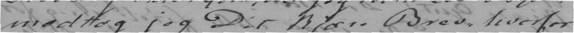modtog jeg Dit kjære Brev, hvorfor
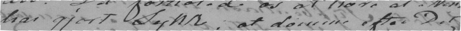har gjort Lykke; at dømme efter Dit
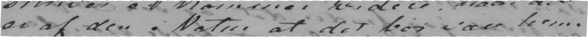er af den Natur at det bør være hem-
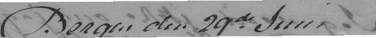Bergen den 29de Juni.
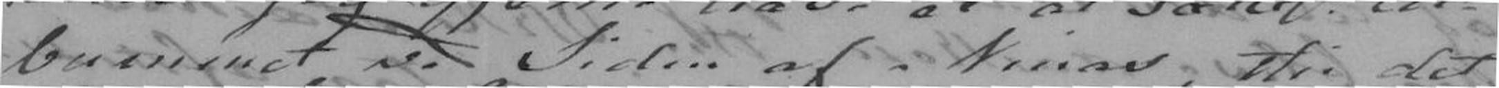bummet ved Siden af Ninas, thi det
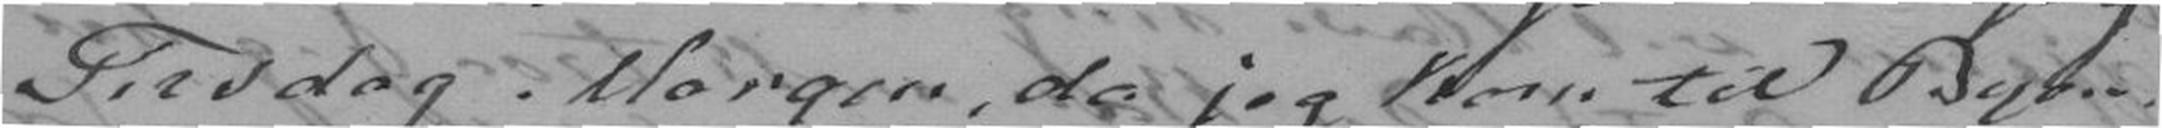Tirsadag Morgen, da jeg kom til Byen
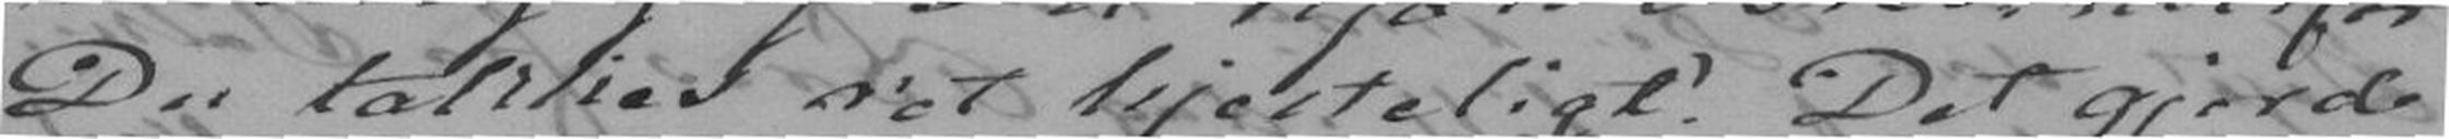Du takkes ret hjerteligt! Det gjorde
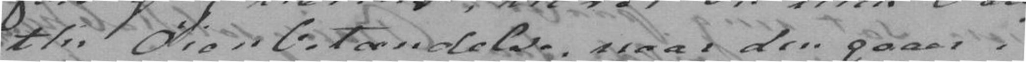thi Øienbetændelse, naar den gaer i
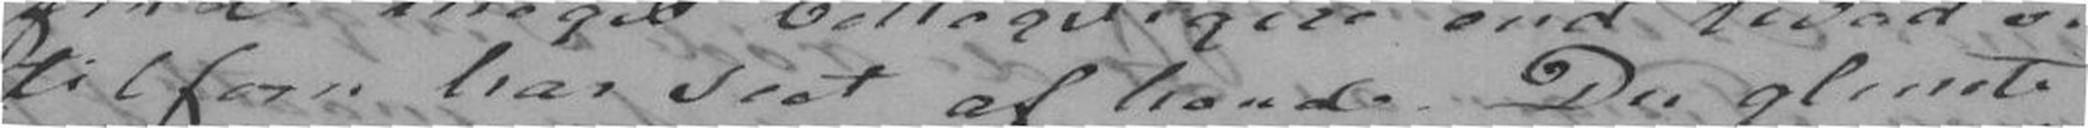tilforn har seet af hende.Du glemte
